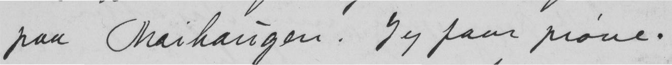paa Maihaungen. Jeg faar prøve.
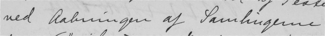ved Aabningen af Samlingerne
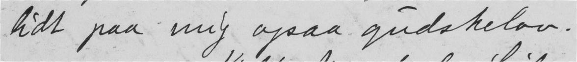lidt paa mig ogsaa gudskelov.
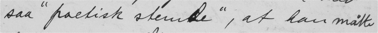saa "poetisk stemde", at han måtte
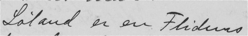Løland er en Flidens
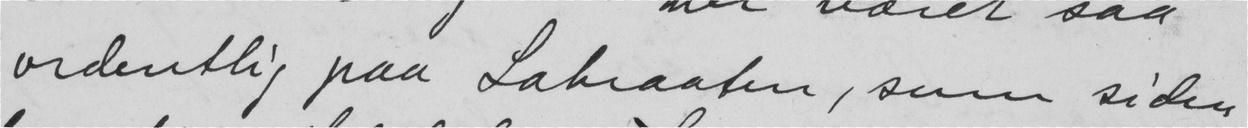ordentlig paa Labraaten, som siden
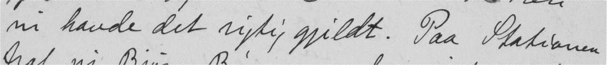vi havde det rigtig gjildt. Paa Stationen
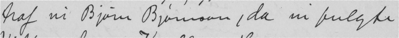traf vi Bjørn Bjørnson, da vi fulgte
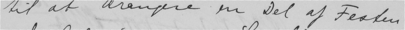til at arengere en Del af Festen
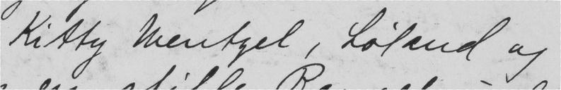Kitty Wentzel, Løland og
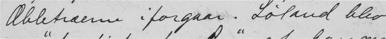Æbletræerne iforgaar. Løland blev
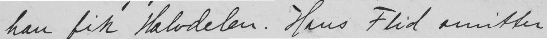han fik Halvdelen. Hans Flid smitter
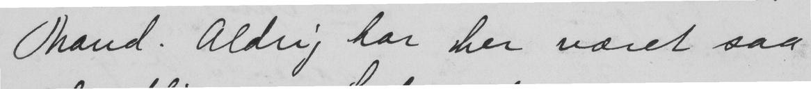Mand. Aldrig har her været saa
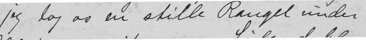jeg tog os en stille Rangel under
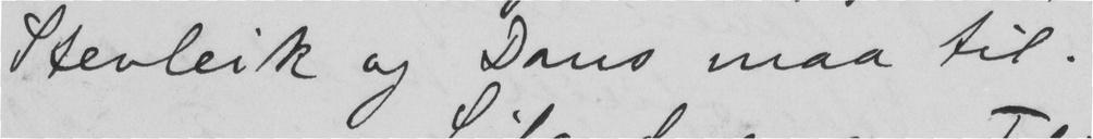Stevleik og Dans maa til.
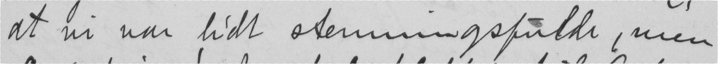at vi var lidt stemningsfulde, men
